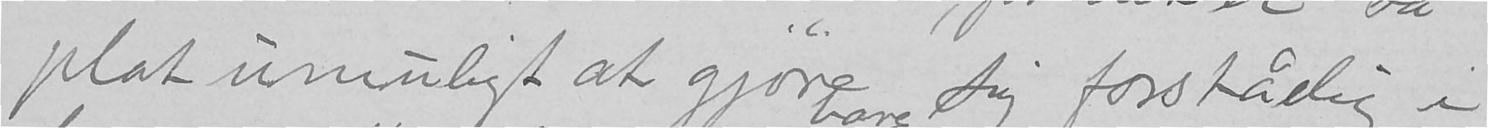plat umuligt at gjøre sig forståelig i
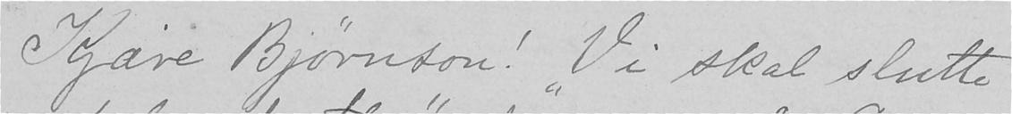Kjære Bjørnson! "Vi skal slutte
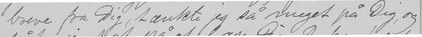breve fra Dig, tænkte jeg så meget på Dig, og
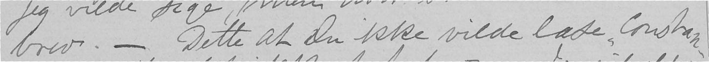brev. - Dette at Du ikke vilde læse "Constan-
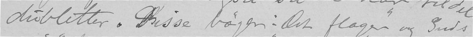dubletter. Disse bøger: "Det flager" og "Guds
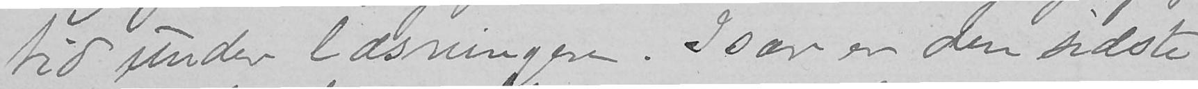tid under læsningen. Især er den sidste
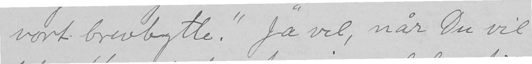vort brevbytte." Ja vel, når Du vil
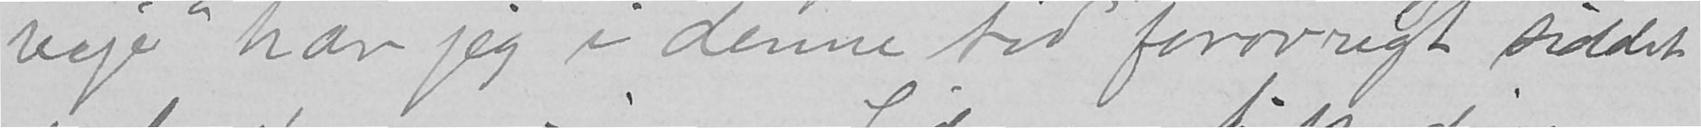veje" har jeg i denne tid forøvrigt siddet
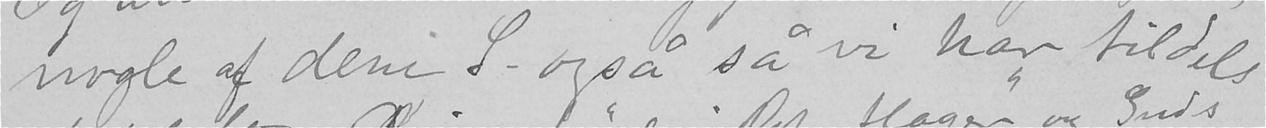nogle af dem S. også så vi har tildels
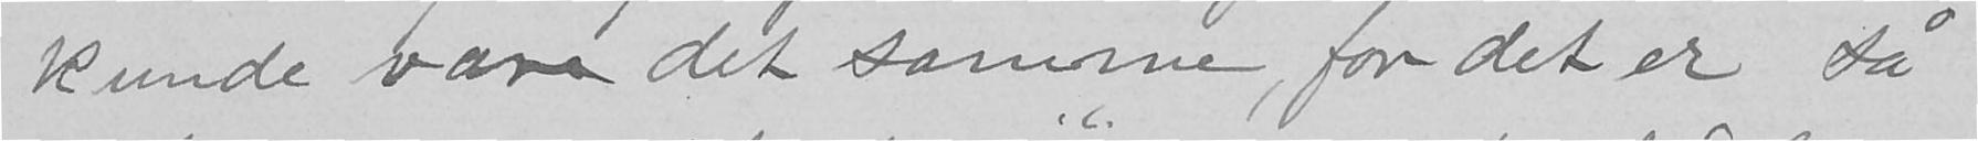kunde være det samme, for det er så
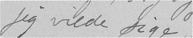jeg vilde sige,
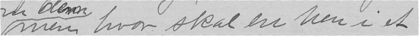men hvor skal en hen i et
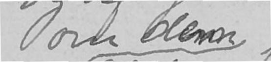om dem
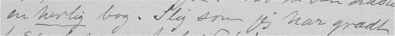en herlig bog. Slig som jeg har grædt
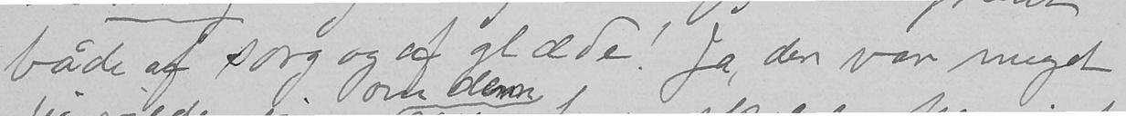både af sorg og af glæde! Ja, der var meget
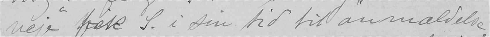veje" fik S. i sin tid til anmældelse.
